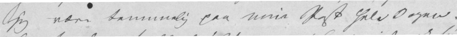jeg være temmelig paa min Post hele Dagen.
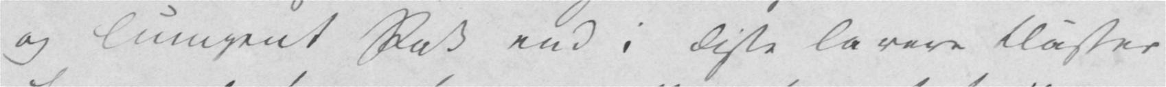og lumpent Pak end i disse lavere Klasser
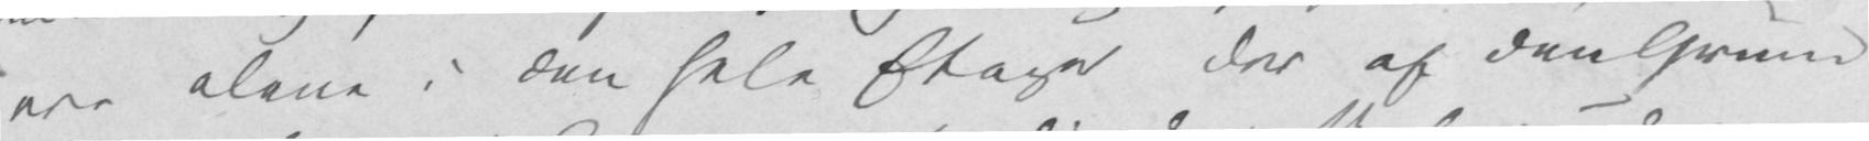ere alene i den hele Etage der af den Grund
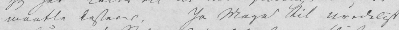maatte kasseres. Ja Mage til uredeligt
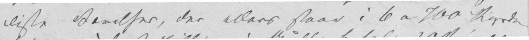disse Værelser, der ellers staae i 6 a 700 Rigsdaler
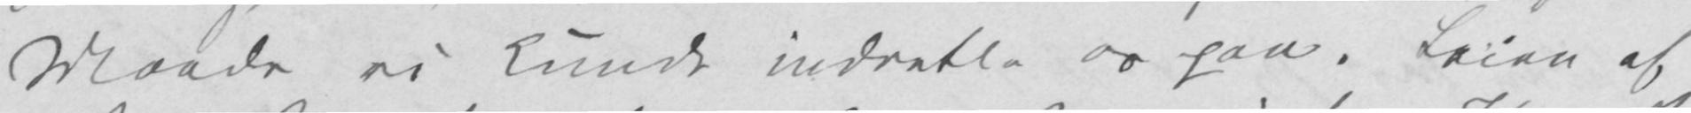Maade vi kunde indrette os paa. Leien af
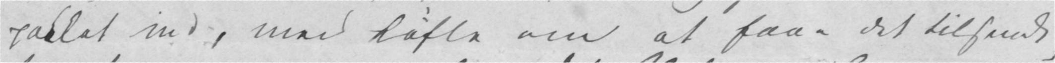pakket ind, med Løfte om at faae det tilsendt,
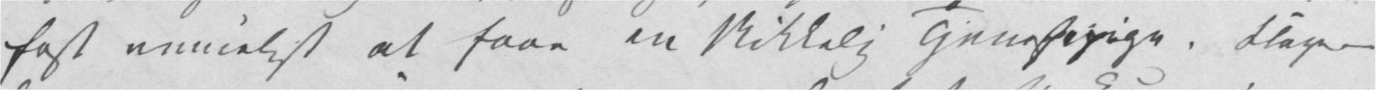fast umueligt at faae en skikkelig Tjenestepige. Klager
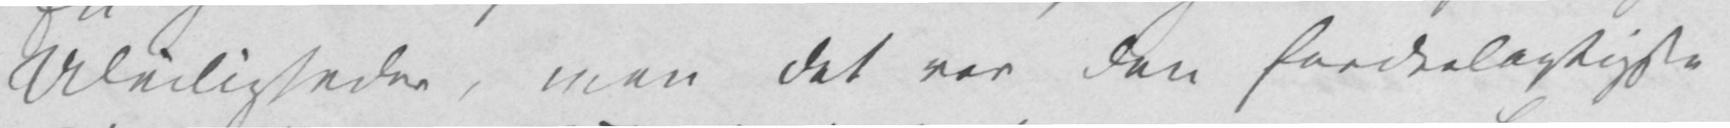Uleiligheder, men det var den fordeelagtigste
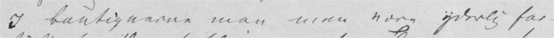i Boutiquerne maa man være yderlig for-
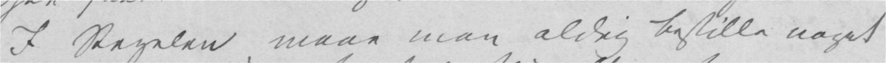I Regelen maae man aldrig bestille noget
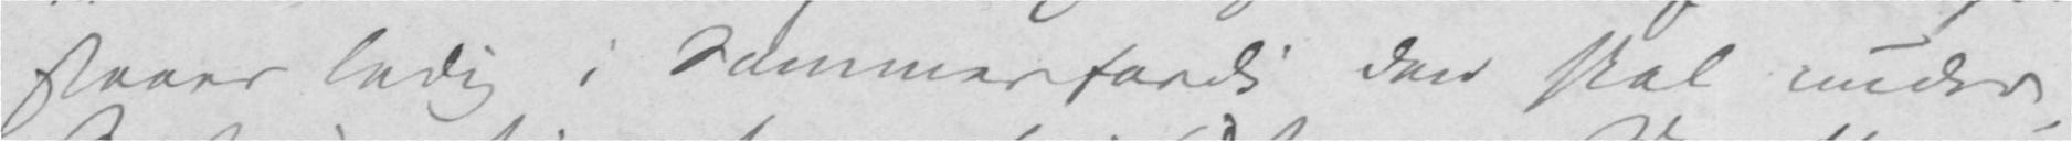staaer ledig i Sommer fordi den skal under
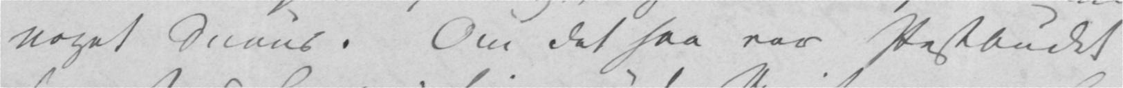noget Snaus. Om det saa var Postbudet,
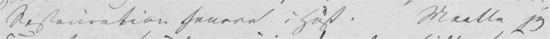Restauration senere i Høst. Maatte jeg
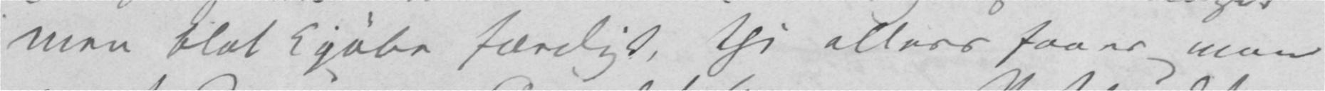men blot kjøbe færdigt, thi ellers faaer man
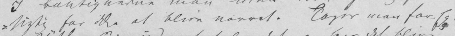sigtig for ikke at blive narret. Tager man for Ex.
